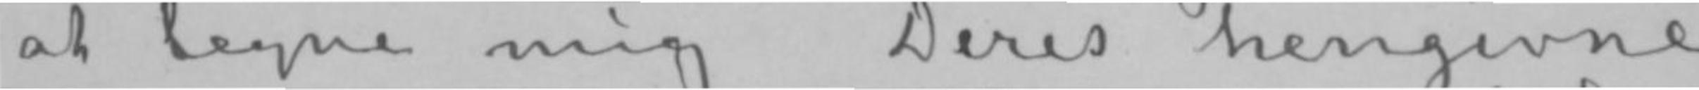at tegne mig Deres hengivne
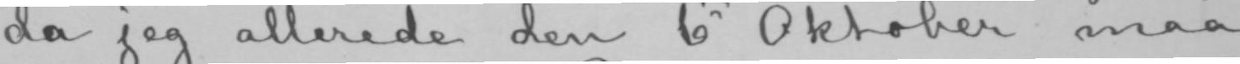da jeg allerede den 6te Oktober maa
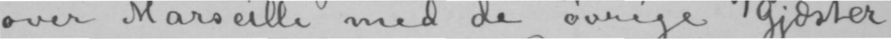over Marseille med de øvrige Gjæster,
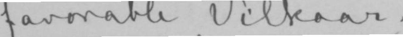favorable Vilkaar.
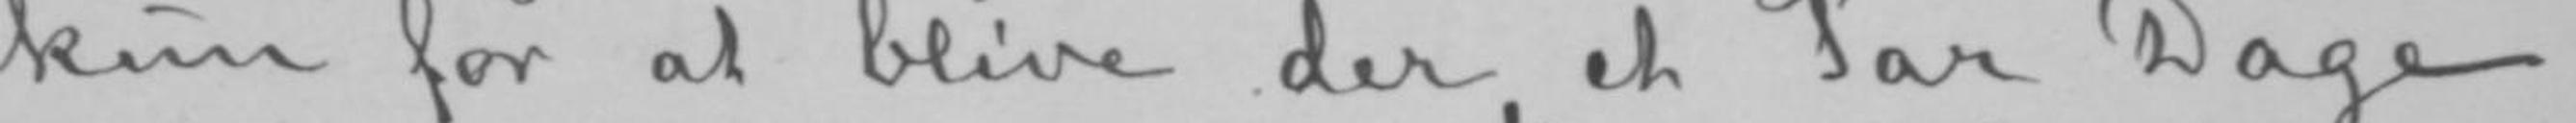kun for at blive der et Par Dage,
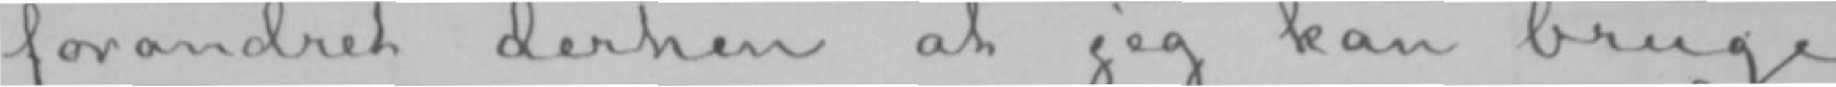forandret derhen at jeg kan bruge
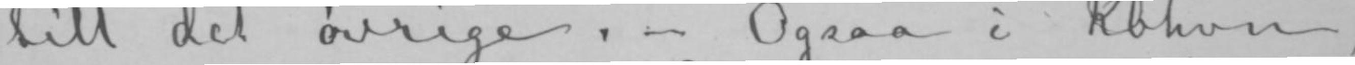till det øvrige.- Ogsaa i Kbhvn,
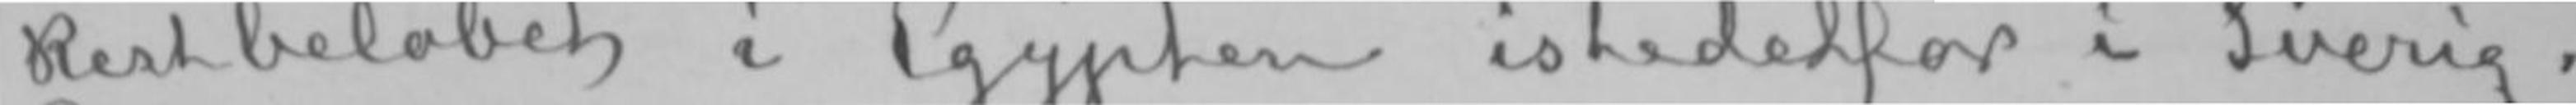Restbeløbet i Egypten istedetfor i Sverig.
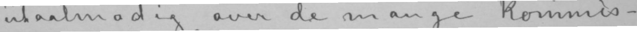utaalmodig over de mange Kommis-
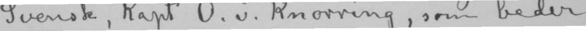Svensk, Kapt O. v. Knorring, som beder
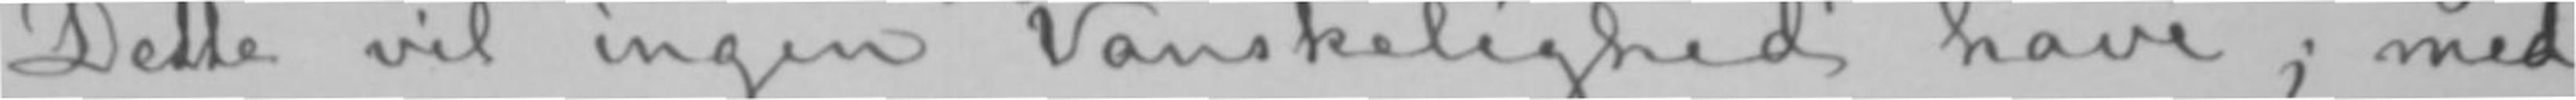Dette vil ingen Vanskelighed have; med
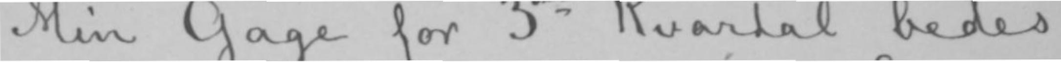Min Gage for 3die Kvartal bedes
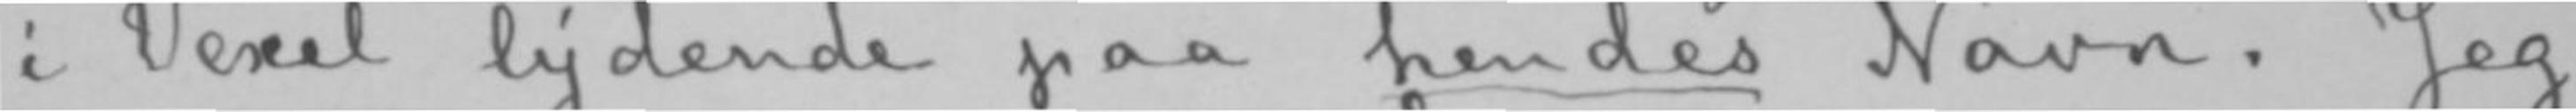i Vexel lydende paa hendes Navn. Jeg
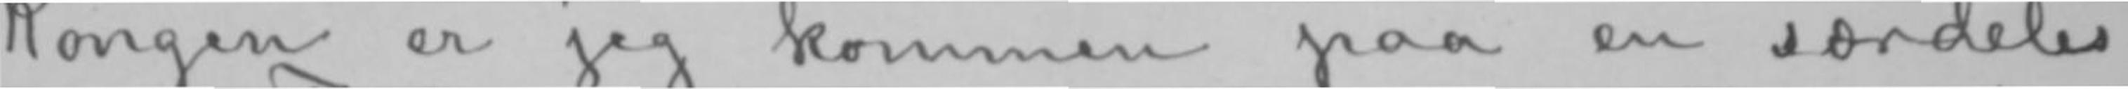Kongen er jeg kommen paa en særdeles
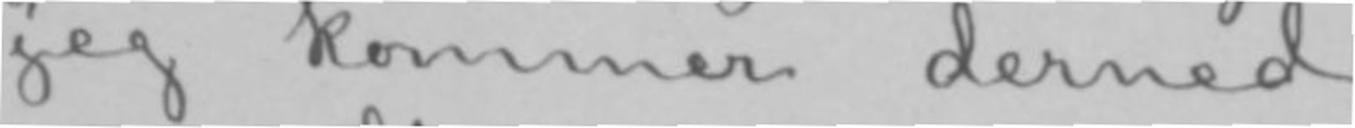jeg kommer derned.
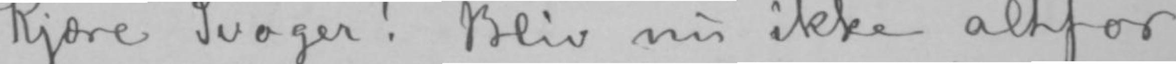Kjære Svoger! Bliv nu ikke altfor
